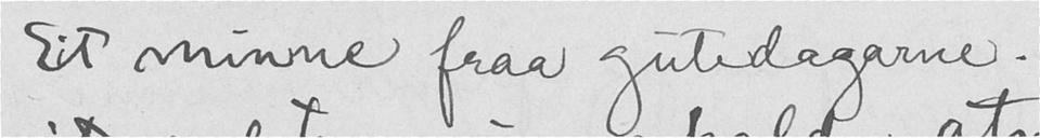Eit minne fraa gutedagarne.
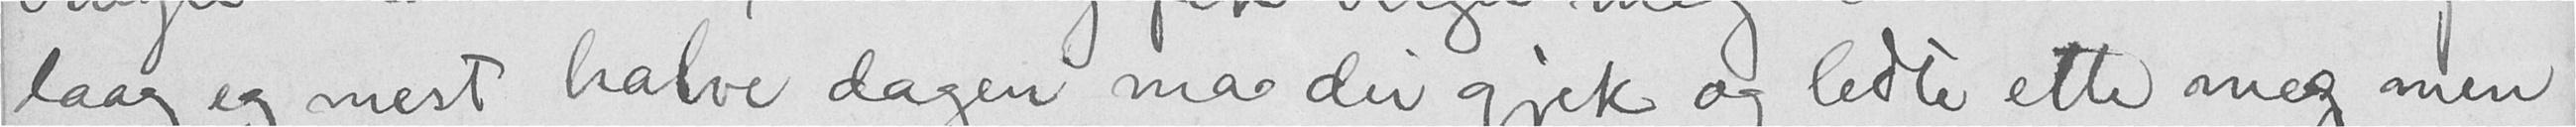laag eg mest halve dagen mas dei gjek og ledte ette meg men
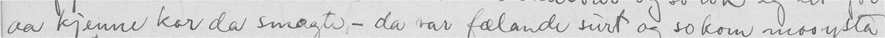aa kjenne kor da smagte, - da var fælande surt og so kom mosysta
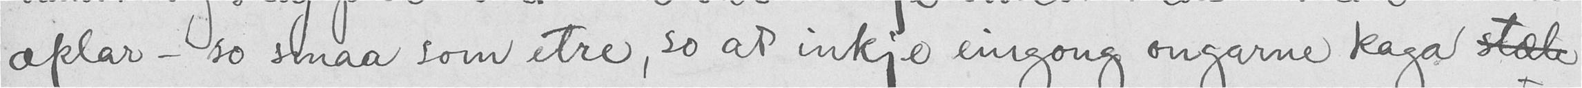aplar - so smaa som etre, so at inkje eingong ongarne kaga
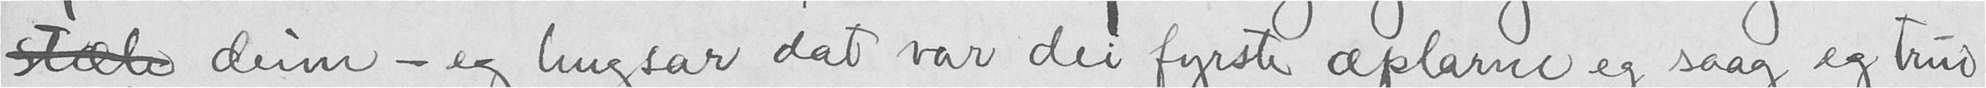deim - eg hugsar det var dei fyrste æplarne er saag eg trud-
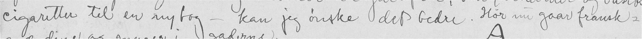cigaretter til en ny bog - kan jeg ønske det bedre. Hør nu gaar fransk-
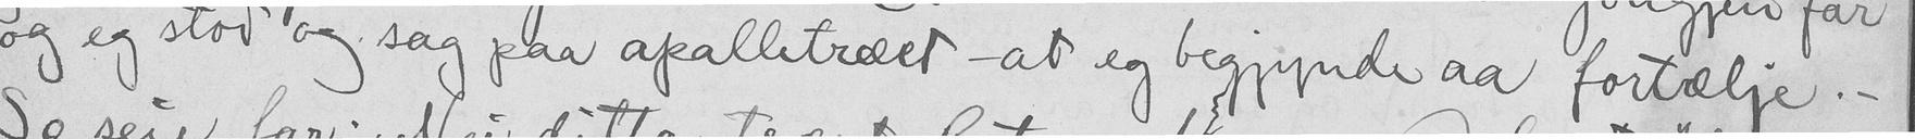og eg stod og sag paa apalletræet at og begjynde aa fortælje. -
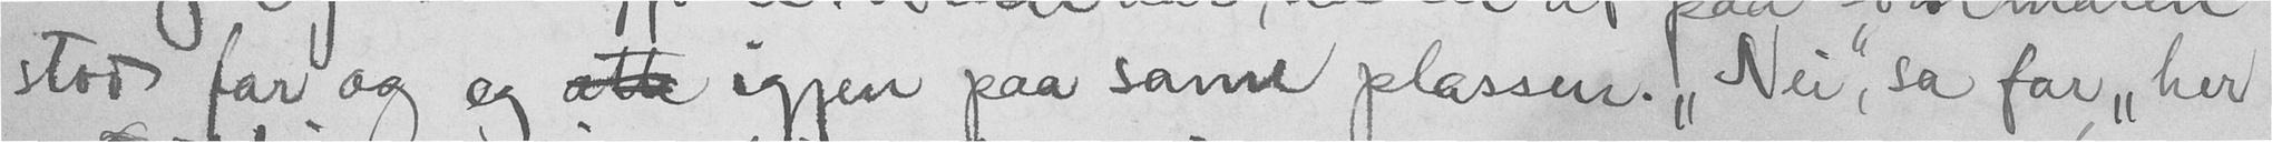stod far og og eg  igjen paa same plassen. "Nei", sa far "her
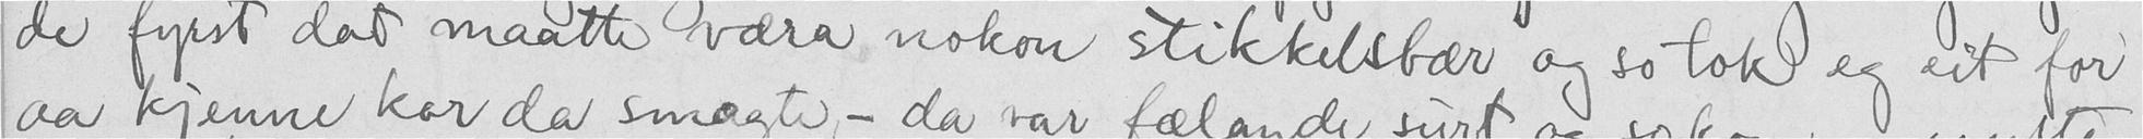de fyrst det maatte væra nokon stikkelsbær og so tok eg eit for
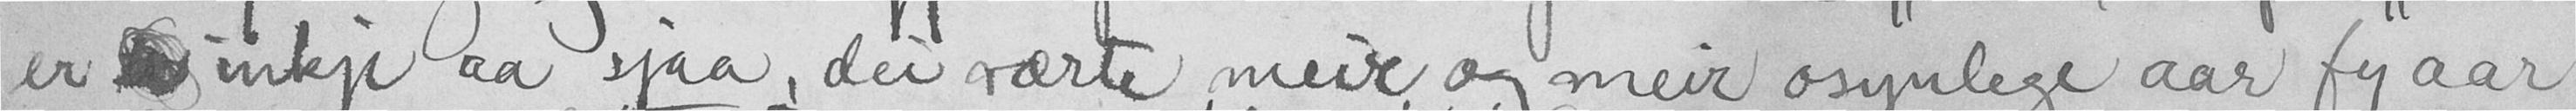er  inkje aa sjaa, dei værte meir og meir osynlege aar fy aar
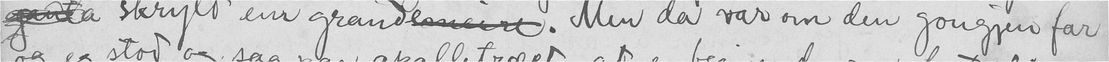skrylt enr grand . Men da var om den gongjen for
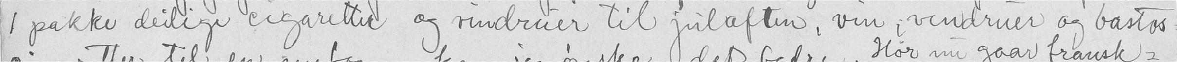1 pakke deilige cigaretter og vindruer til julaften, vin vindruer og bastos-
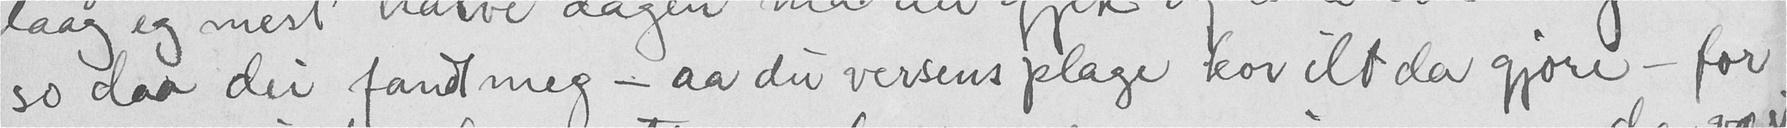so daa dei fandt meg - aa du versens plage kor ilt da gjore - for
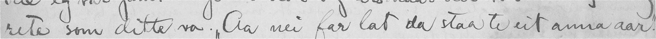rete som dette va. "Aa nei far lat da staa te eit ania aar."
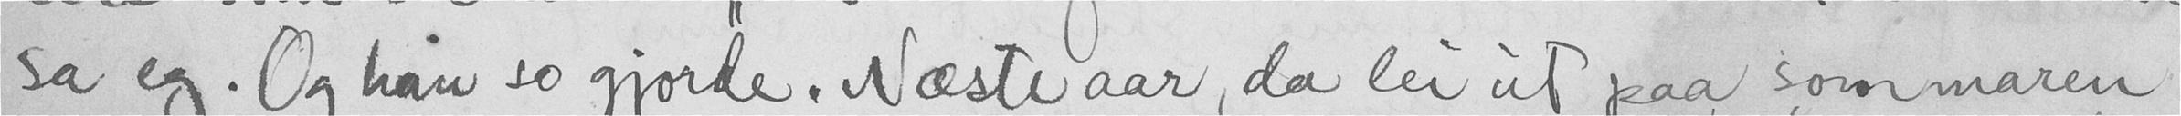sa eg. Og han so gjorde. Næste aar, da lei ut paa sommaren
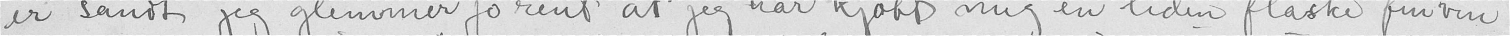er sandt jeg glemmer jo rent at jeg har kjøbt mig en liden flaske fin vin
